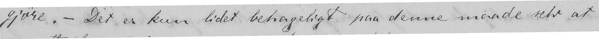gjøre. - Det er kun lidet behageligt paa denne maade selv at
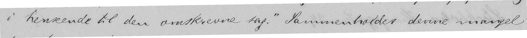i henseende til den omskrevne sag". Sammenholdes denne mangel
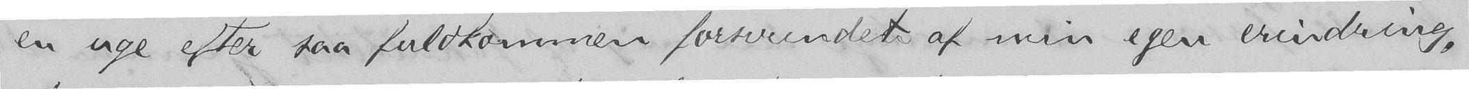en uge efter saa fuldkommen forsvundet af min egen erindring,
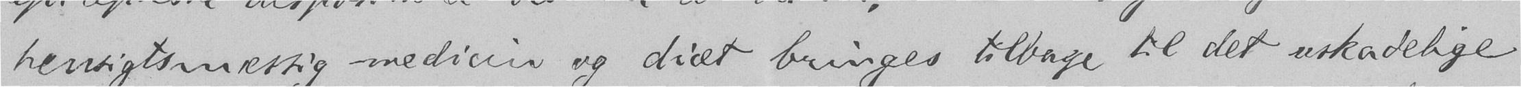hensigtsmæssig medicin og diæt bringes tilbage til det uskadelige
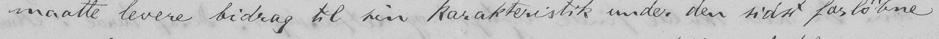maatte levere bidrag til sin karakteristik under den sidst forløbne
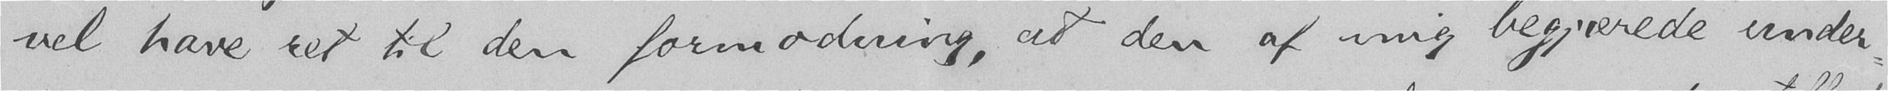vel have ret til den formodning, at den af mig begjærede under-
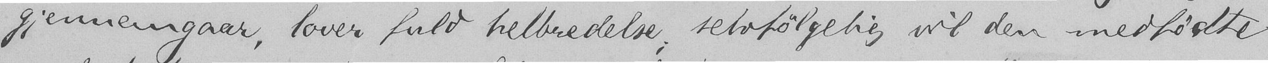gjennemgaar, lover fuld helbredelse; selvfølgelig vil den medfødte
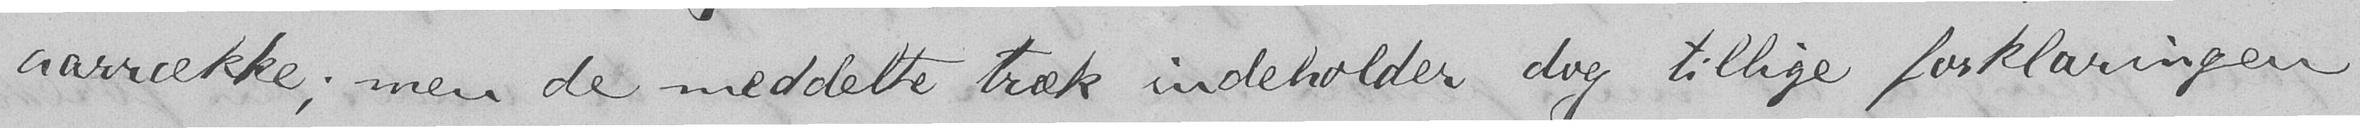aarrække; men de meddelte træk indeholder dog tillige forklaringen
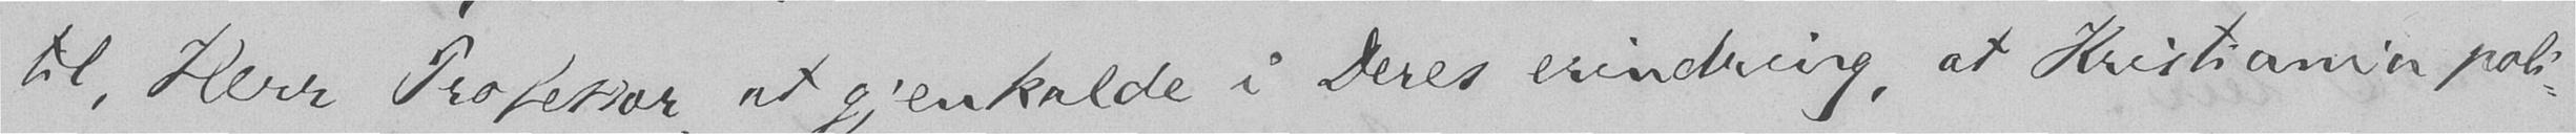til, Herr Professor, at gjenkalde i Deres erindring, at Kristiania poli-
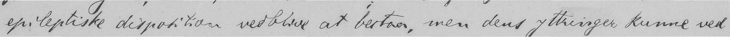epileptiske disposition vedblive at bestaa, men dens yttringer kunne ved
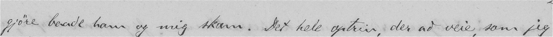gjøre baade ham og mig skam. Det hele optrin, der ad veie, som jeg
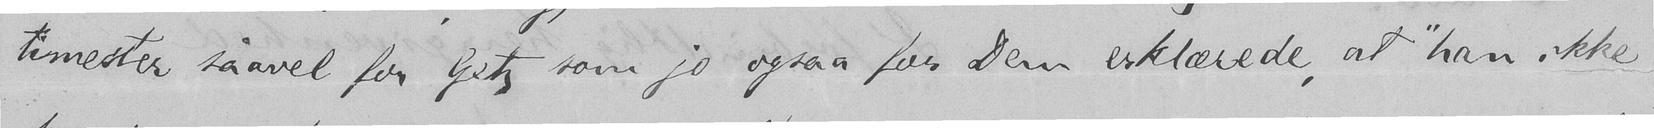timester saavel for Getz som jo ogsaa for Dem erklærede, at "han ikke
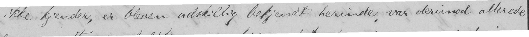ikke kjender, er bleven adskillig bekjendt herinde, var derimod allerede
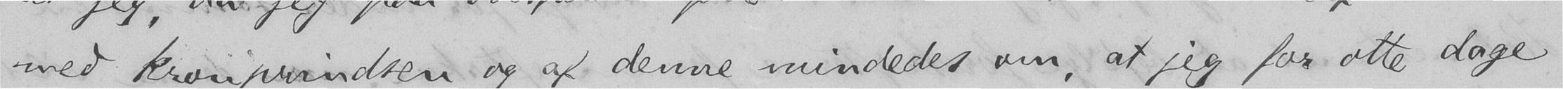med kronprindsen og af denne mindedes om, at jeg for otte dage
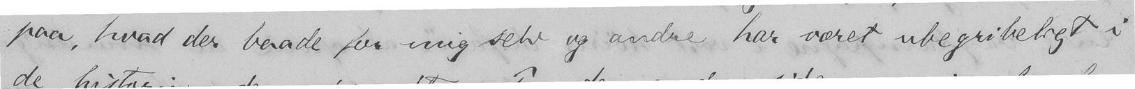paa, hvad der baade for mig selv og andre har været ubegribeligt i
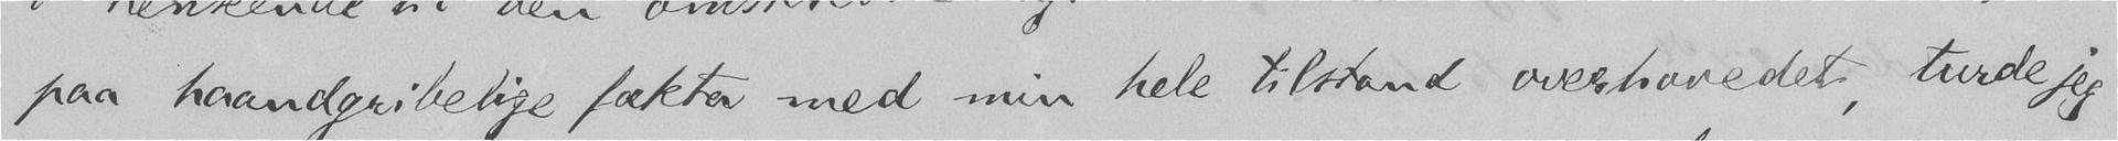paa haandgribelige fakta med min hele tilstand overhovedet, turde jeg
